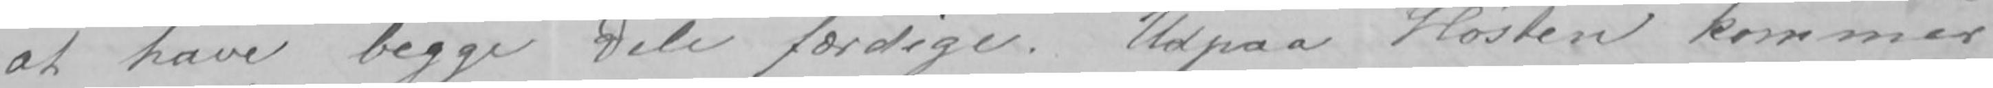at have begge Dele færdige. Udpaa Høsten kommer
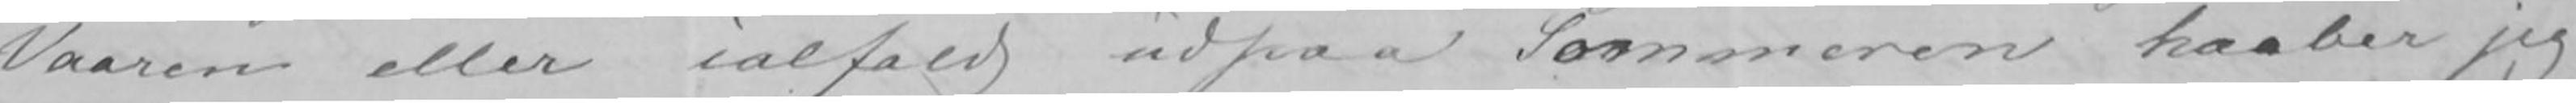Vaaren eller ialfald udpaa Sommeren haaber jeg
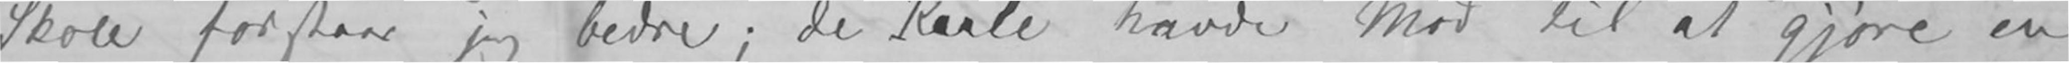Skole forstaar jeg bedre; de Karle havde Mod til at gjøre en
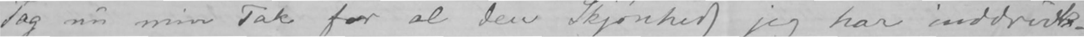Tag nu min Tak for al den Skjønhed jeg har inddruk-
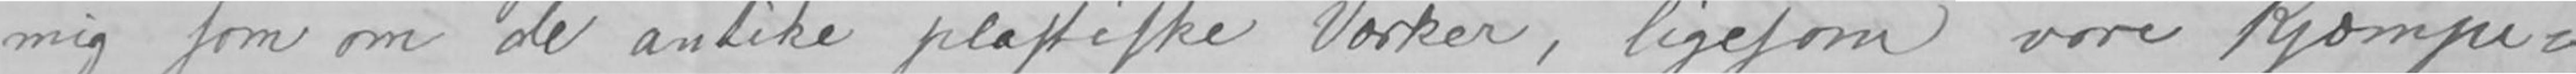mig som om de antike plastiske Værker, ligesom vore Kjæmpe-
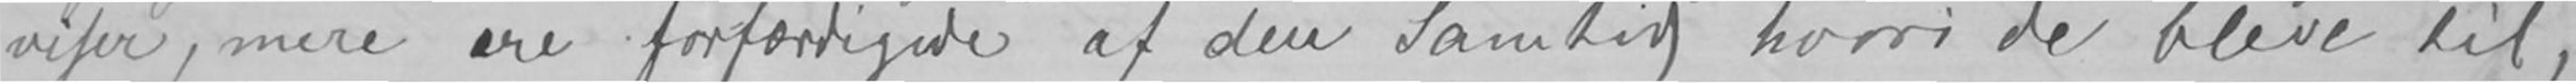viser, mere ere forfærdigede af den Samtid hvori de bleve til,
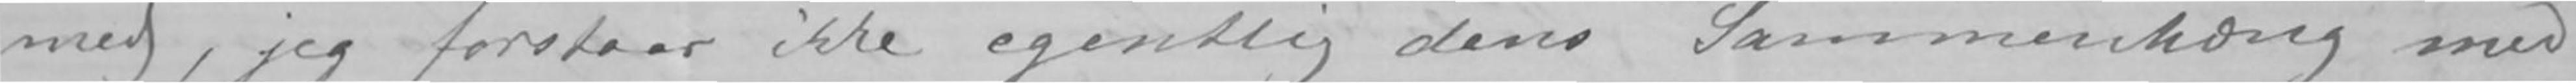med, jeg forstaar ikke egentlig dens Sammenhæng med
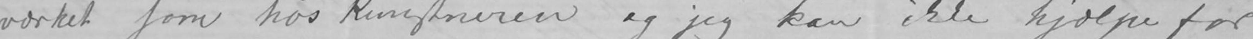værket som hos Kunstneren og jeg kan ikke hjælpe for
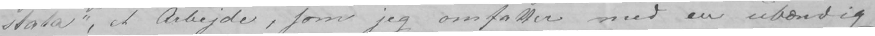stata», et Arbejde, som jeg omfatter med en ubændig
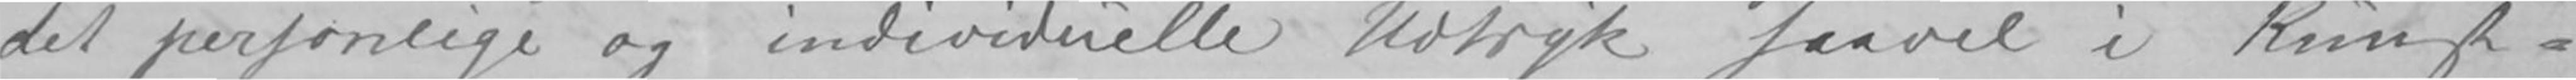det personlige og individuelle Udtryk saavel i Kunst-
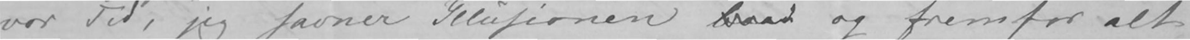vor Tid, jeg savner Illusionen baad og fremfor alt
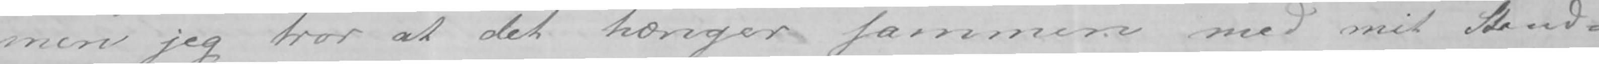men jeg tror at det hænger sammen med mit Stand-
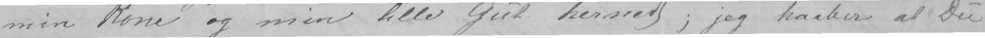min Kone og min lille Gut herned; jeg haaber at Du
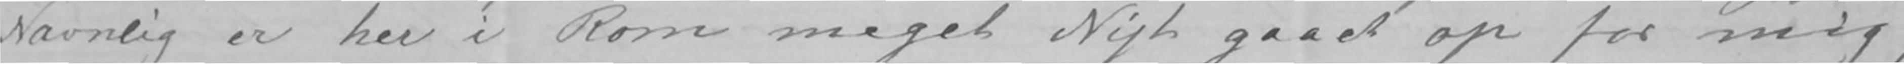Navnlig er her i Rom meget Nyt gaaet op for mig;
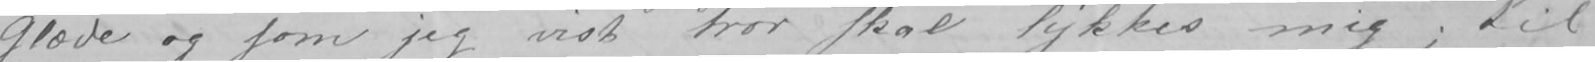Glæde og som jeg vist tror skal lykkes mig; til
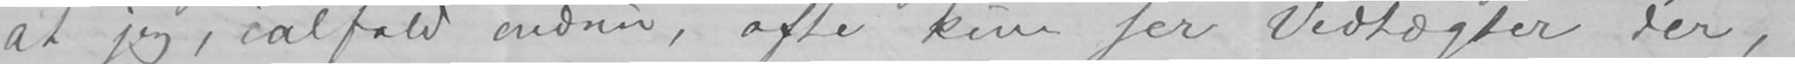at jeg, ialfald endnu, ofte kun ser Vedtægter der,
